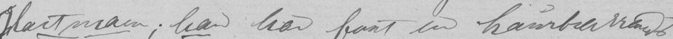Hartmann; han har faat en Laurbærkrands
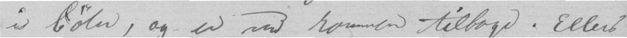i Cøln, og er nu kommen tilbage. Ellers
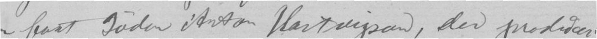faat Jøden Anton Hartvigson, der producere-
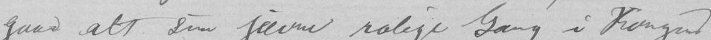gaar alt sin jævne rolige Gang i Kongens
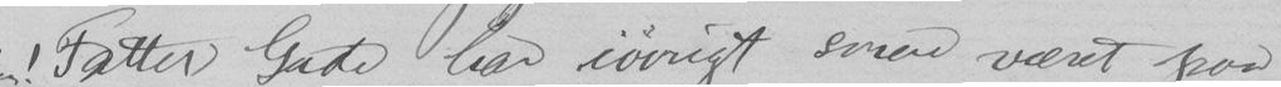Fatter Gade har iøvrigt senere været paa
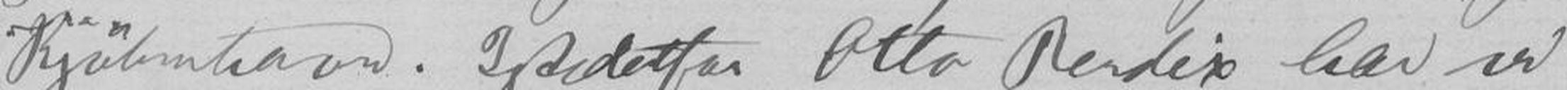Kjøbenhavn. Istedetfor Otto Bendix har vi
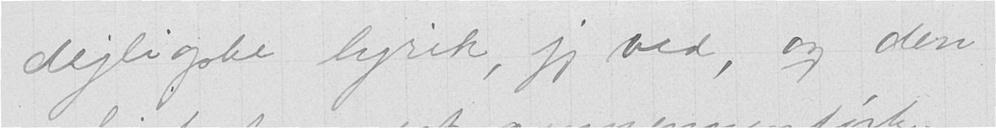deiligste lyrik, jeg ved, og den
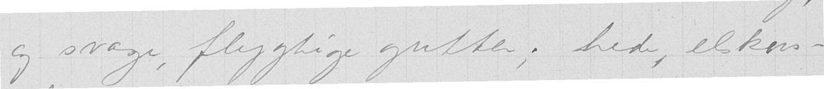og svage, flygtige gutter; hede, elskovs-
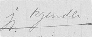jeg kjender.
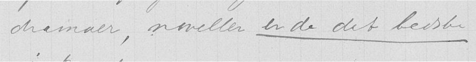dramaer, noveller er de det bedste
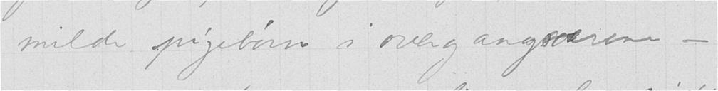milde pigebørn i overgangsalder —
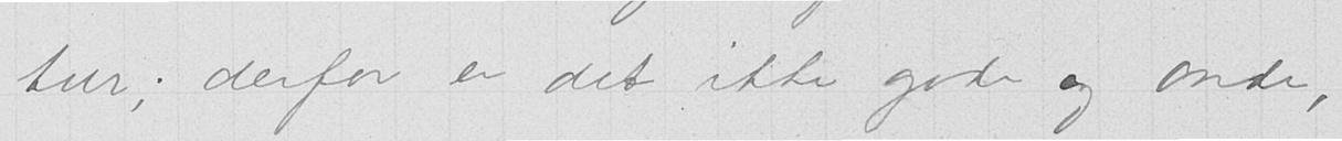ratur; derfor er det ikke gode og onde,
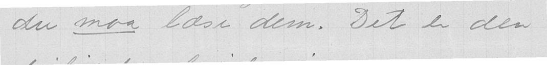du maa læse dem. Det er den
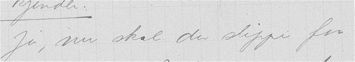Ja, nu skal du slippe for
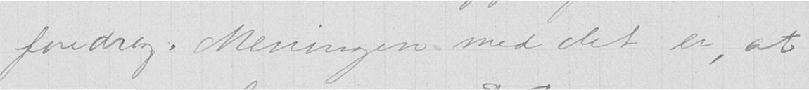foredrag. Meningen med det er, at
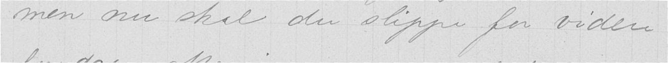men nu skal du slippe for videre
